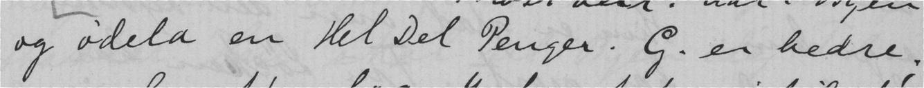og ødela en Hel Del Penger. G. er bedre;
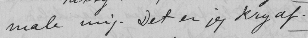male mig. Det er jeg Kry af.
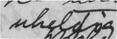uheldig
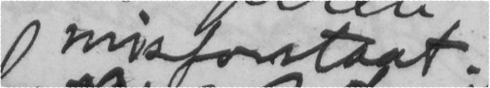misforstaat.
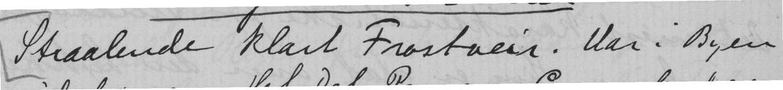Straalende klart Frostveir. Var i Byen
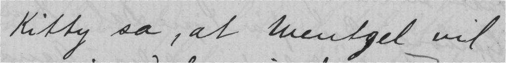Kitty sa, at Wentzel vil
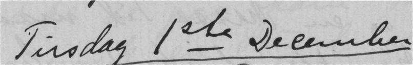Tirsdag 1ste December
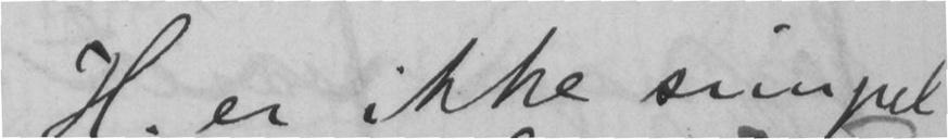H. er ikke simpel
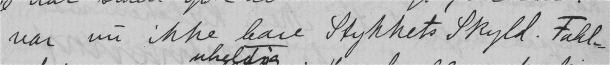var nu ikke bare Stykkets Skyld. Fahl-
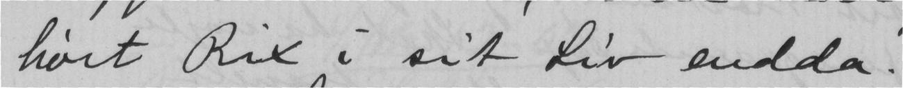hørt Rix i sit Liv endda.
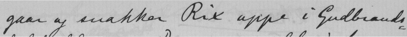gaar og snakker Rix oppe i Gudbrands-
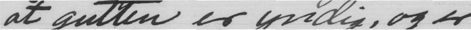at gutten er yndig, og er
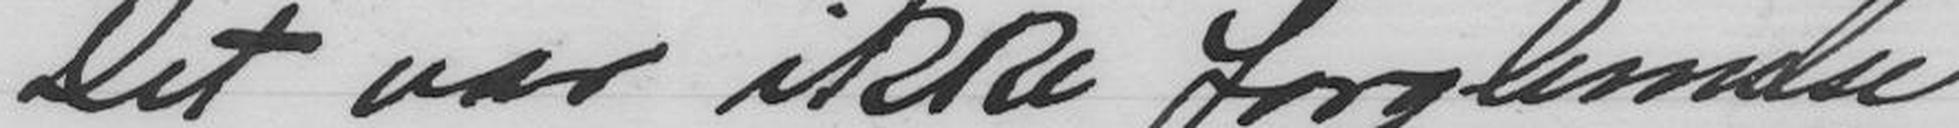Det var ikke forglemelse
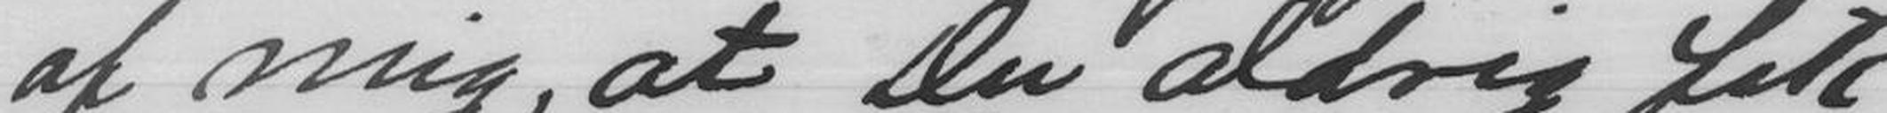af mig, at Du aldrig fik
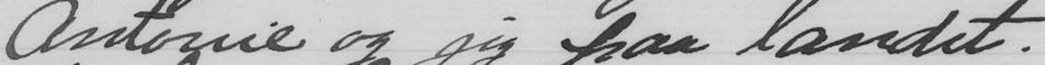Antonie og jeg paa landet.
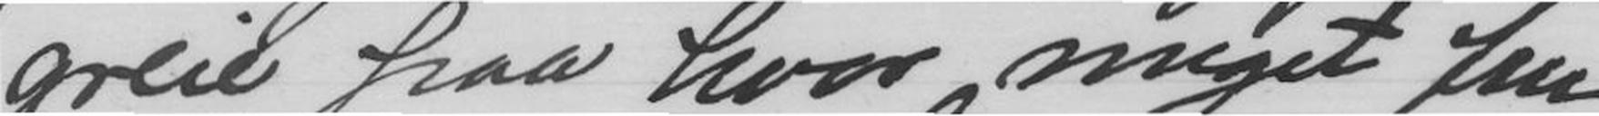greie paa hvor meget fru
